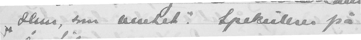"Hun, som ventet." Spekulerer på
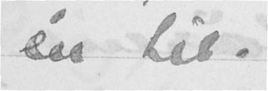en til.
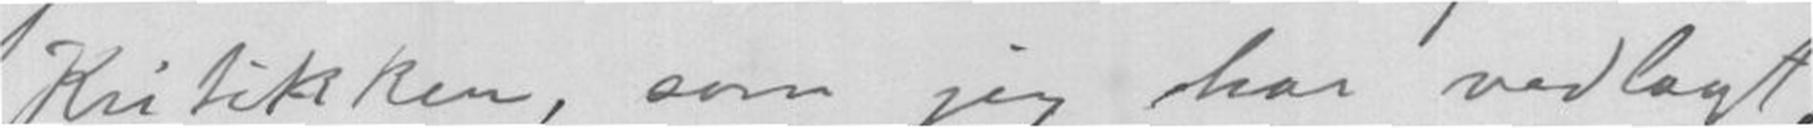Kritikken, som jeg har vedlagt,
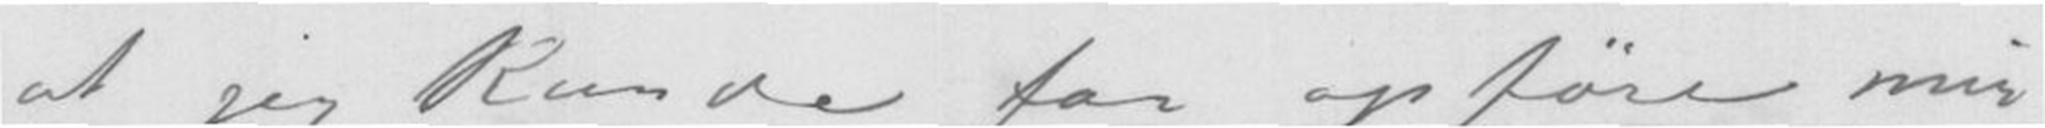at jeg Kunde faa opføre min
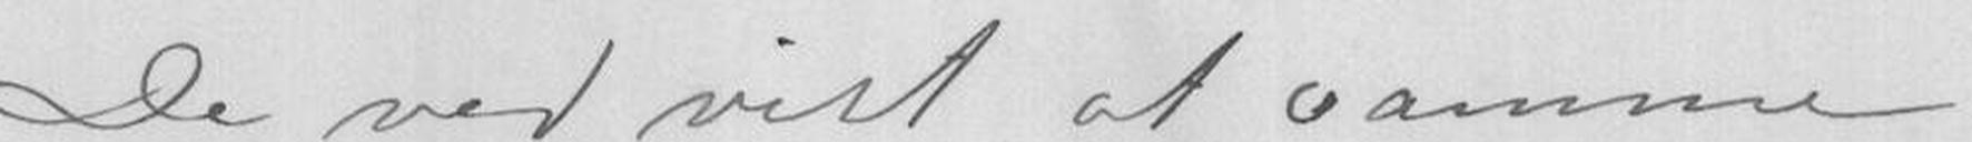De ved vist at samme
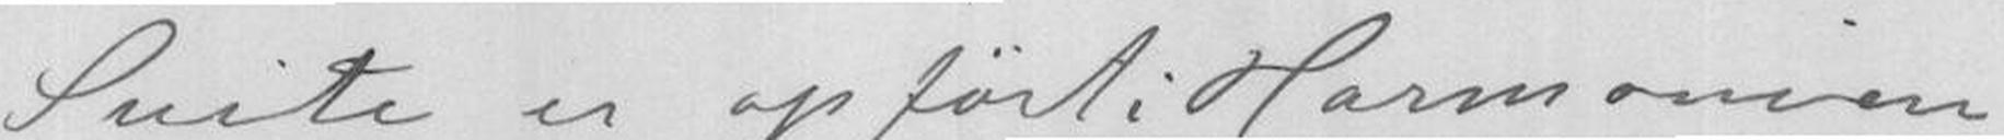Suite er opført i Harmonien
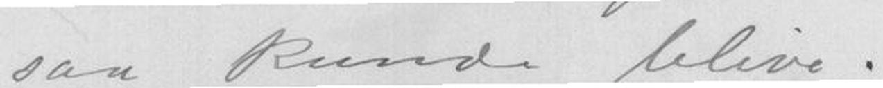saa kunde blive.
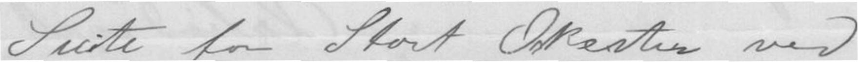Suite for Stort orkester ved
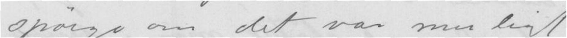spørge om det var muligt
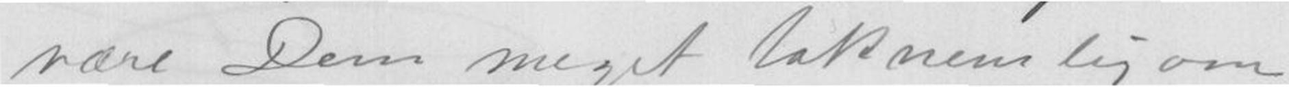være Dem meget Taknemlig om
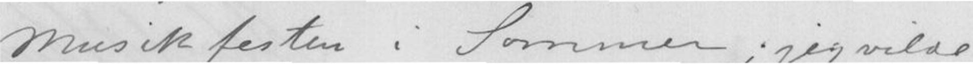musikfesten i Sommer; jeg vilde
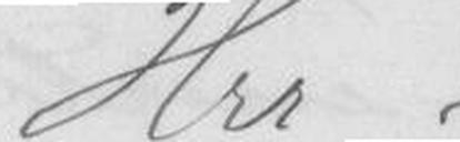Hrr
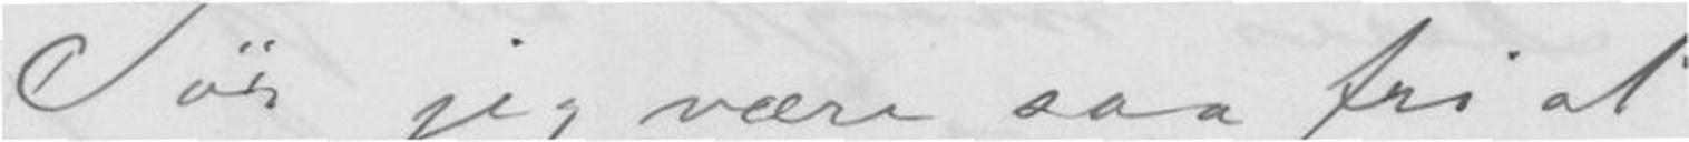Tør jeg være saa fri at
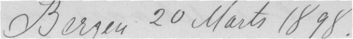Bergen 20 Marts 1898
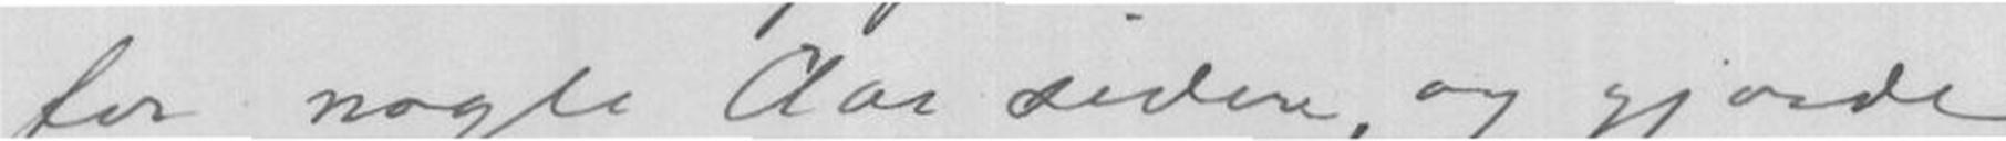for nogle Aar siden, og gjorde
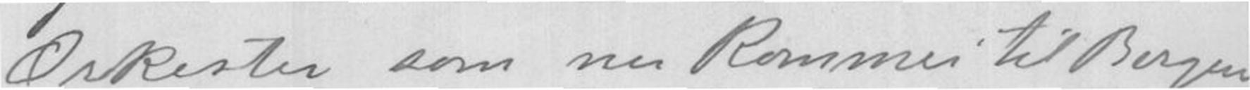Orkester som nu kommer til Bergen,
